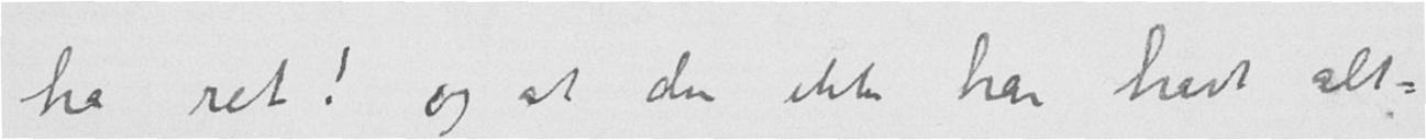ha ret! og at du ikke har havt alt-
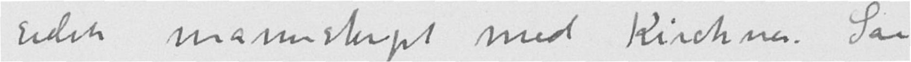sidste manuskript med Kirchner. Saa
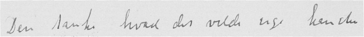Den tanke hvad det vilde sige kanske
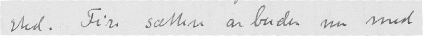sted. Fire sættere arbeider nu med
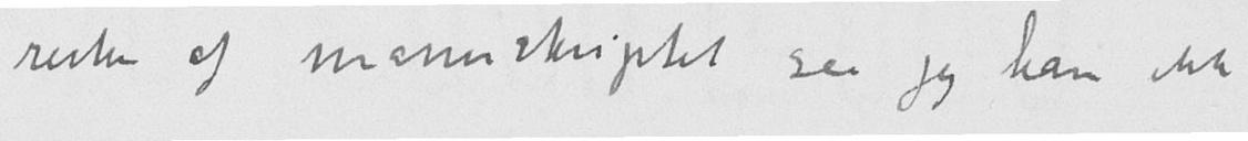resten af manuskriptet saa jeg kan ikke
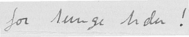for tunge tider!
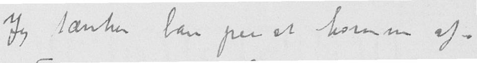Jeg tænker bare paa at komme af-
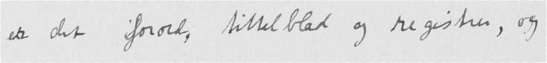et det forord, tittelblad og register, og
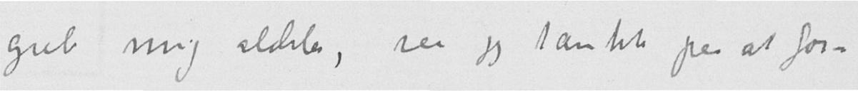greb mig aldeles, saa jeg tænkte paa at for-
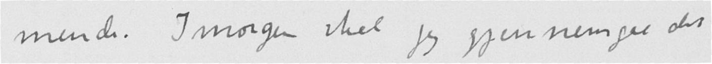mende. I morgen skal jeg gjennemgaa det
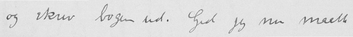og skrev bogen ud. Gid jeg nu maatte
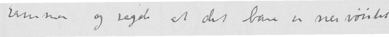sammen og sagde at det bare er nervøsitet
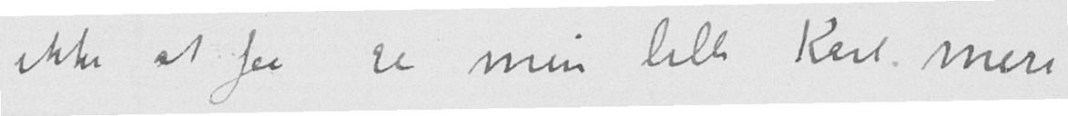ikke at faa se min lille Karl mere
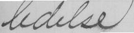ledelse.
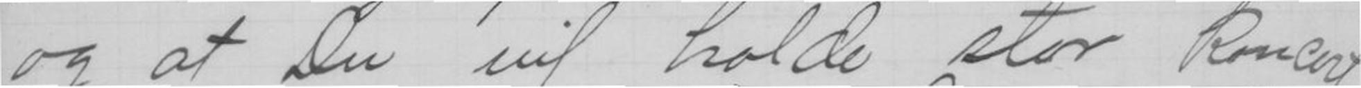og at Du vil holde stor koncert
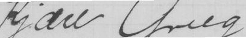Kjære Grieg!
